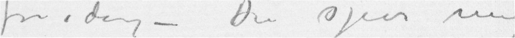for idag – Du spør mig
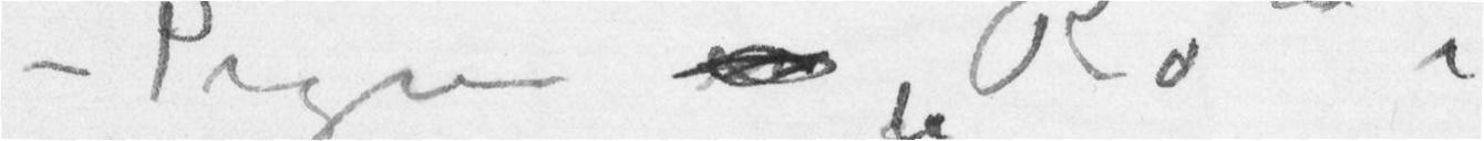– Pigen«Ro» i
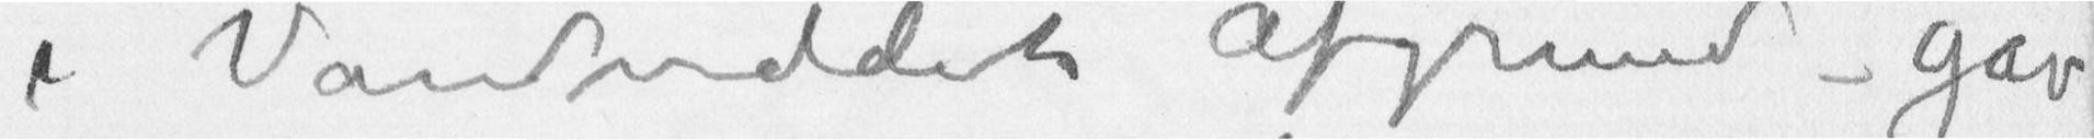i Vandviddets Afgrund – gav
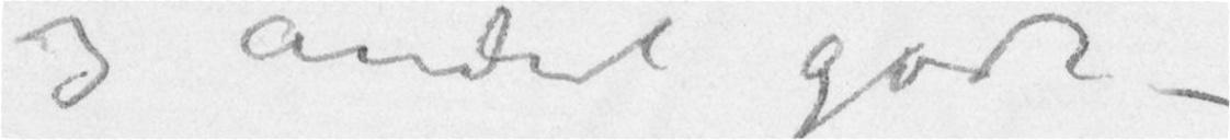og andet godt –
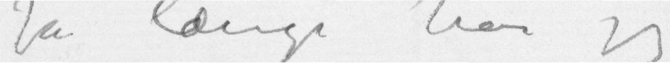Ja længe har jeg
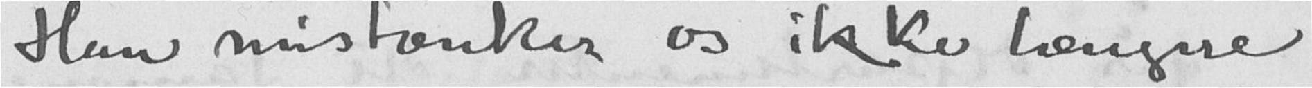Han mistænker os ikke længere
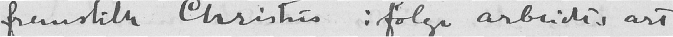fremstille Christus ifølge arbeidets art
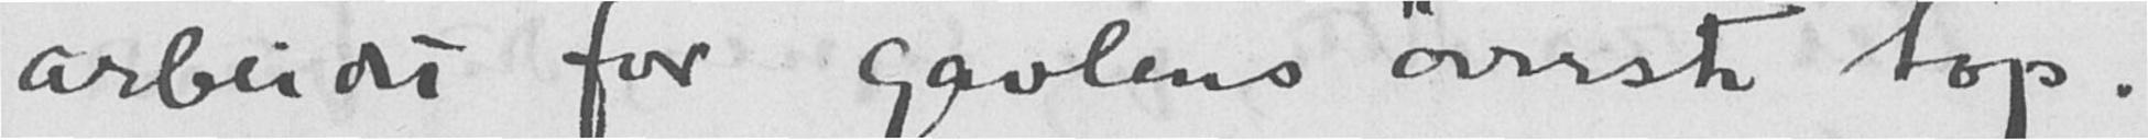arbeidet for gavlens øverste top.
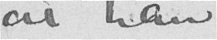at han
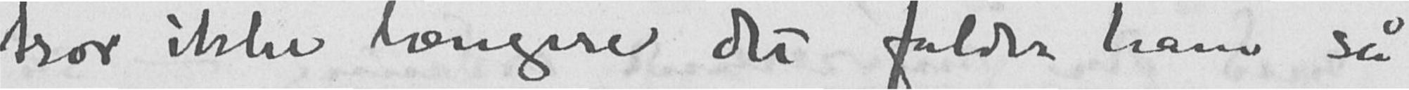tror ikke længere det falder ham så
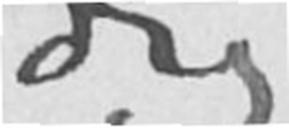dig
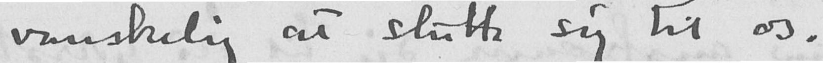vanskelig at slutte sig til os.
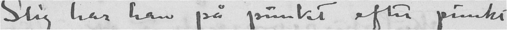Slig har han på punkt efter punkt
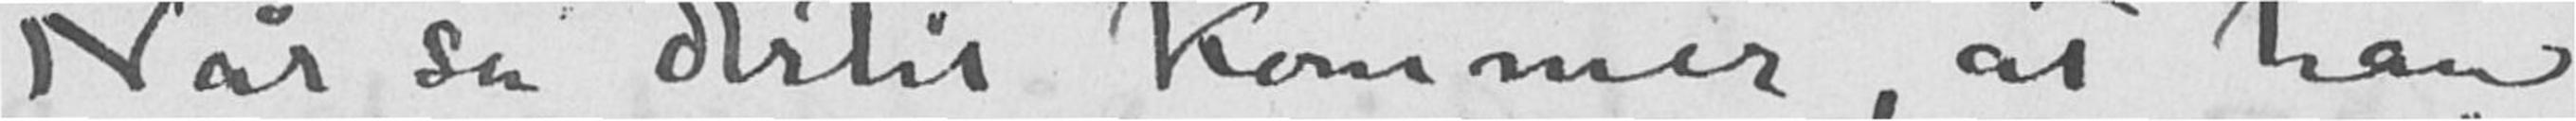Når sá dertil kommer, at han
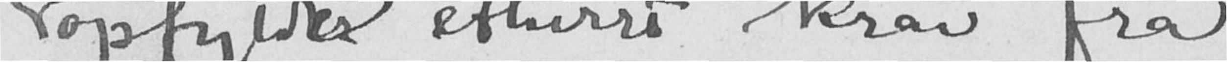opfylde ethvert krav fra
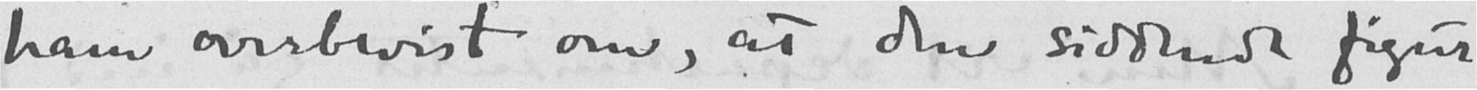ham overbevist om, at den siddende figur
